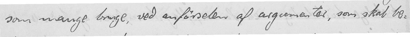som mange bruge, ved anførselen af argumenter, som skal be-
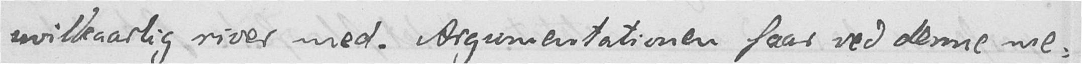uvilkaarlig river med. Argumentationen faar ved denne me-
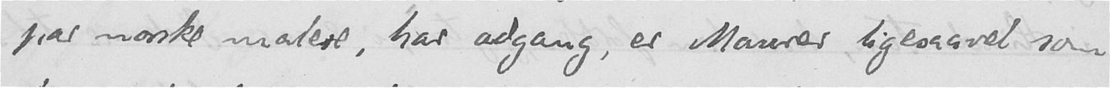par norske malere, har adgang, er Maurer ligesaavel som
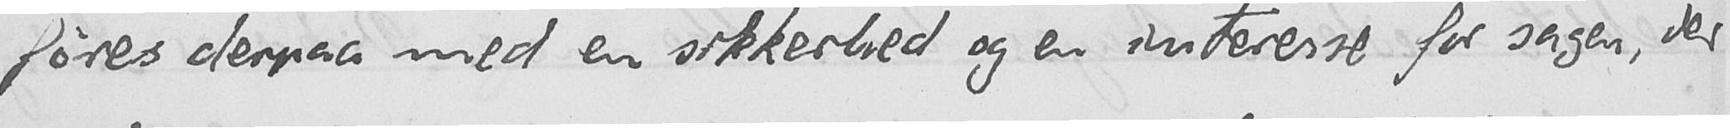føres derpaa med en sikkerhed og en interesse for sagen, der
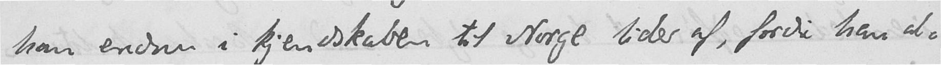han endnu i kjendskaben til Norge lider af, fordi han al-
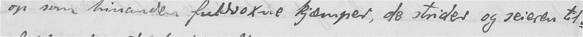op som hinanden fuldvoxne kjæmper, de strider og seieren til-
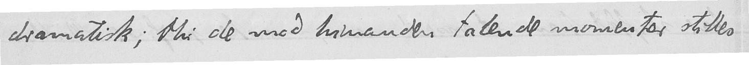dramatisk; thi de med hinanden talende momenter stilles
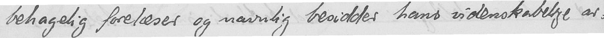behagelig forelæser og navnlig besidder hans videnskabelige ar-
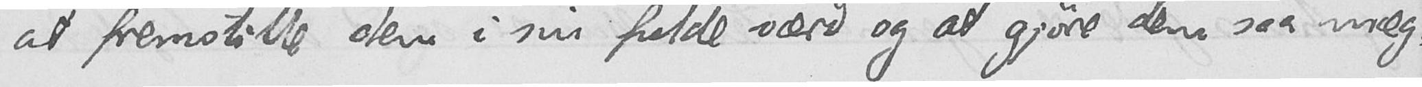at fremstille dem i sin fulde værd og at gjøre dem saa mæg-
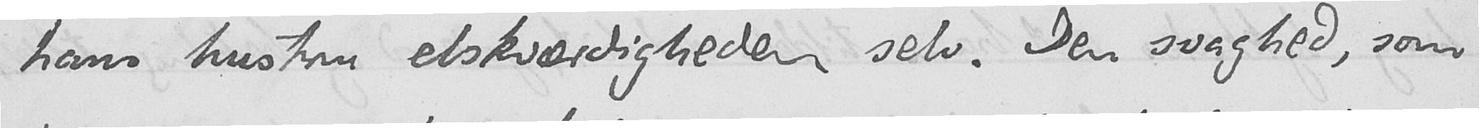hans hustru elskværdigheden selv. Den svaghed, som
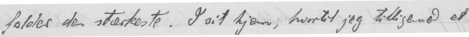falder den stærkeste. I sit hjem, hvortil jeg tilligemed et
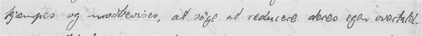kjæmpes og modbevises, at søge at reducere deres egen overtalel-
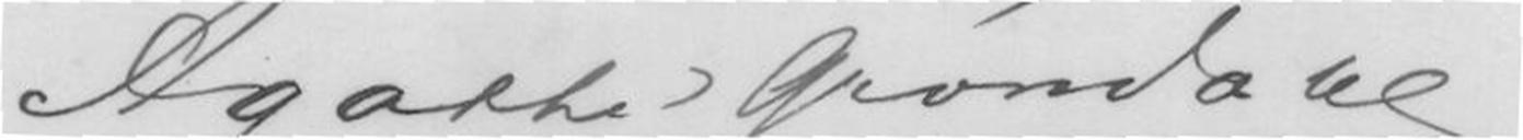Agathe Grøndahl
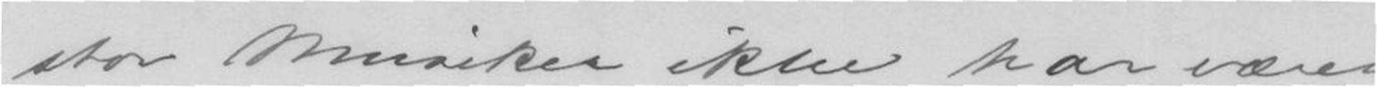stor Musiker ikke har været
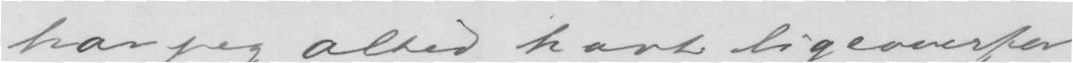har jeg altid havt ligeoverfor
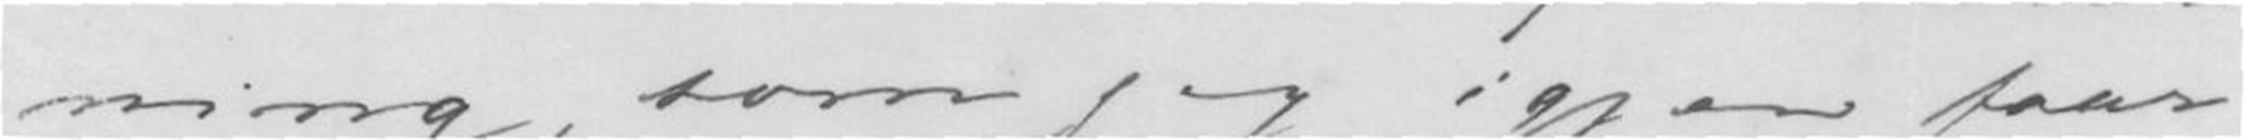ning, som jeg igjen faar
